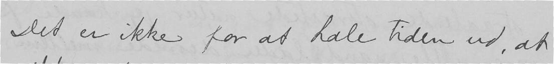Det er ikke for at hale tiden ud, at
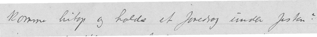komme hitop og holde et foredrag under festen?
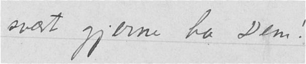svært gjerne ha Dem!
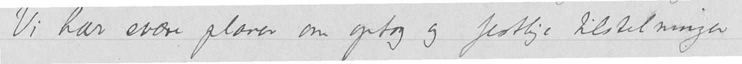Vi har svære planer om optog og festlige tilstelninger
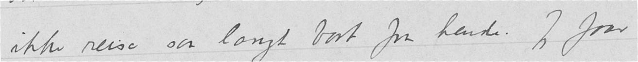ikke reise saa langt bort fra hende. Jeg faar
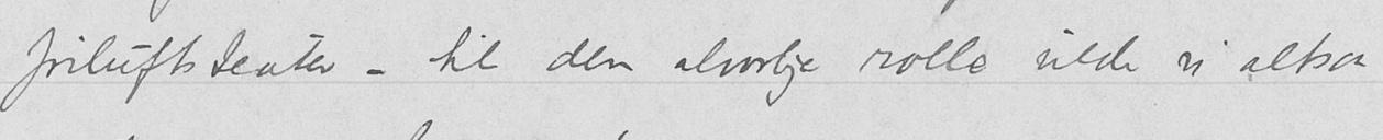friluftsteater - til den alvorlige rolle vilde vi altsaa
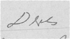Deres
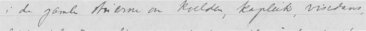i de gamle stuerne om kvelden, kapleik, visedans,
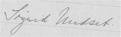Sigrid Undset.
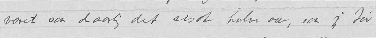været saa daarlig det sisste halve aar, saa jeg tør
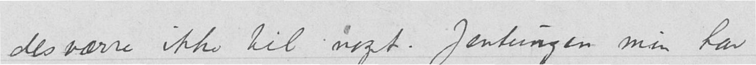desværre ikke til noget. Jentungen min har
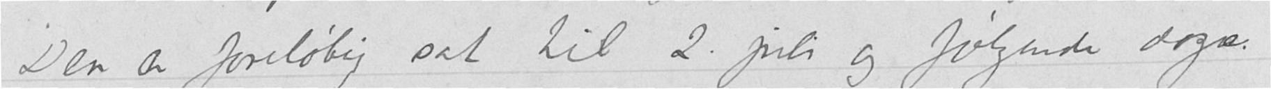Den er foreløbig sat til 2. juli og følgende dager
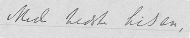Med bedste hilsen,
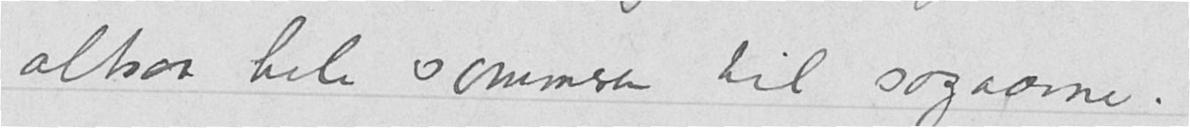altsaa hele sommeren til sagaærne.
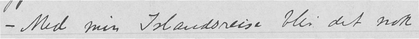– Med min Islandsreise blir det nok
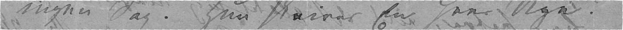ingen Sag. Hun skriver En hver Uge.
